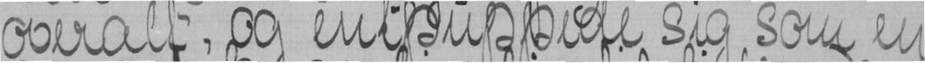overalt og entpuppede sig som en
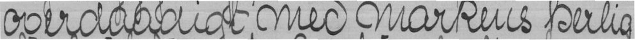overdaadigt med markens herlig
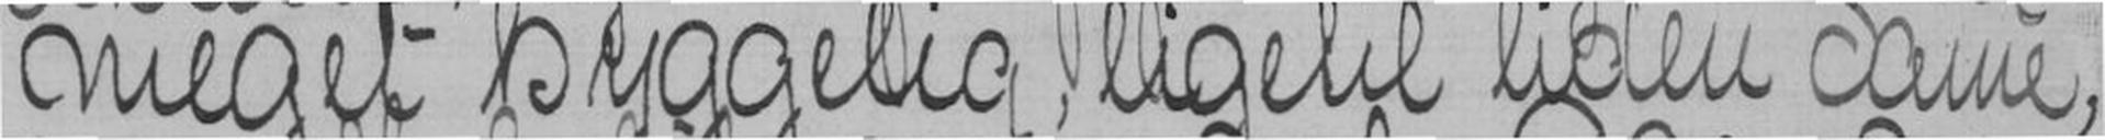meget hyggelig, ligetil liden Dame,
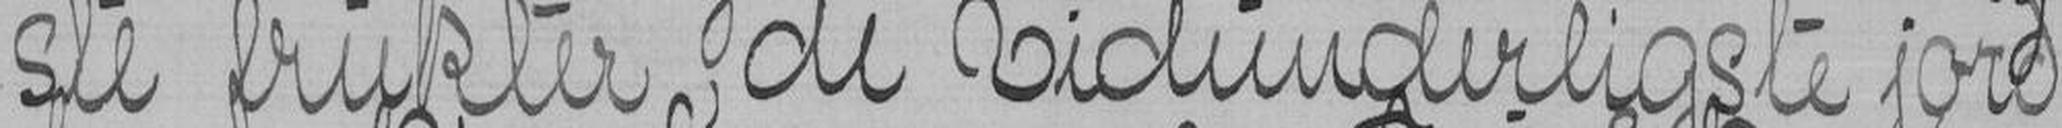ste frukter, de vidunderligste jord-
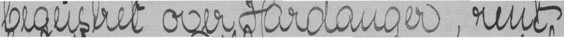begeistret over Hardanger, rent
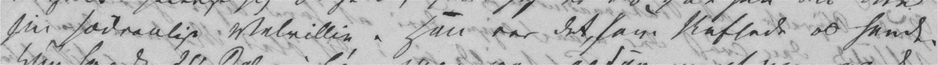sin sædvanlige Velvillie. Han var det, som skaffede os hende.
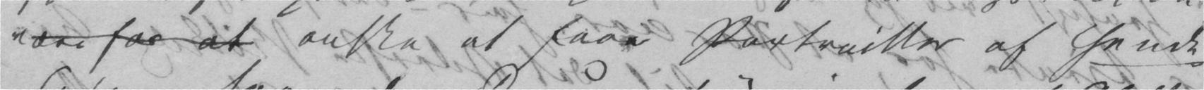være for at ønske at faae Portraitter af hende.
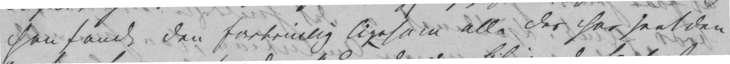han fandt den fortrinlig ligesom alle der har seet den
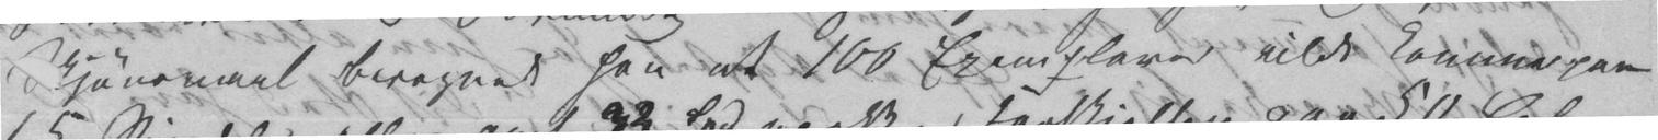Skjønsmaal beregnede han at 100 Exemplarer vilde komme paa
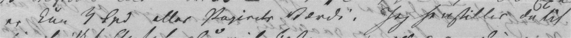er kun 3 Spd eller Papirets Værdi. Jeg henstiller da til
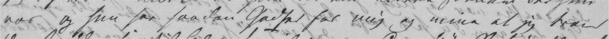var og hun har saadan Godhed for mig og mine at jeg troer
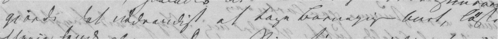gjorde det nødvendigt at tage Barnepigen bort, løste
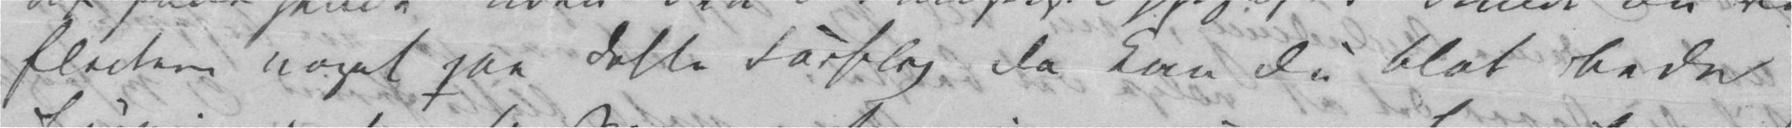flectere noget paa dette Forslag da kan Du blot bede
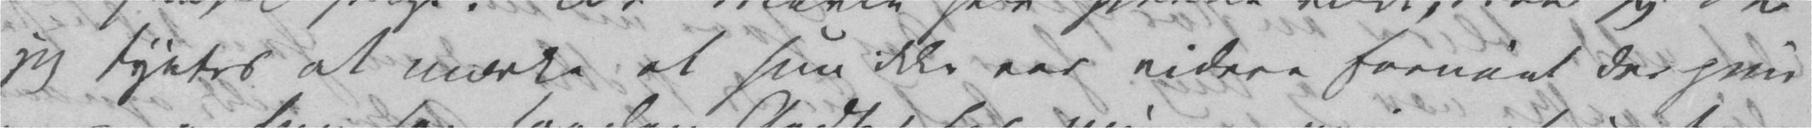jeg syntes at mærke at hun ikke var videre fornøiet der hun
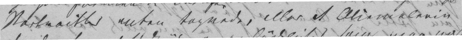Portraitter enten tegnede, eller et Oliemalerie
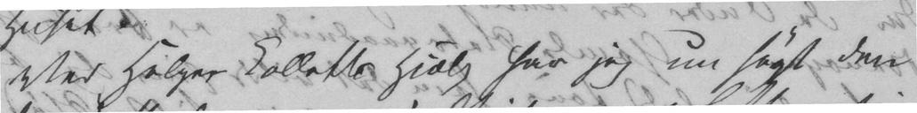Ved Holger Colletts Hjælp har jeg nu søgt den
